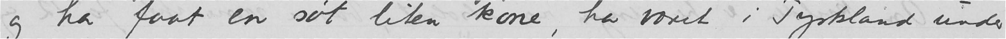og har faat en søt liten kone, har været i Tyskland under
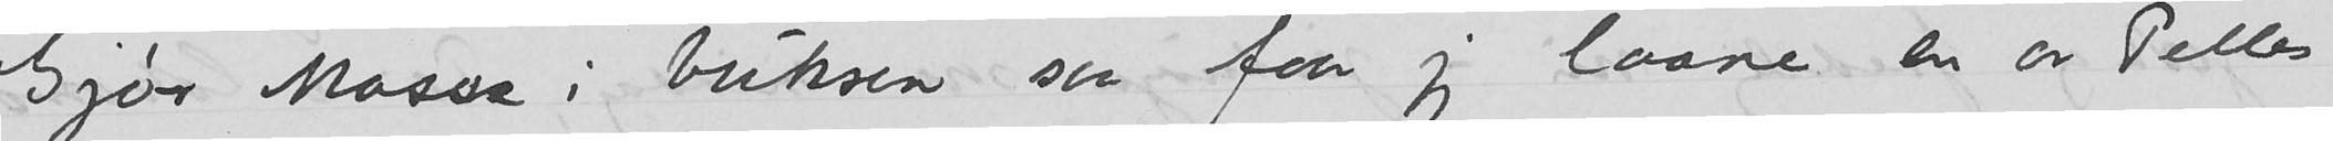Gjør Mosse i buksen saa faar jeg laane en av Pelles
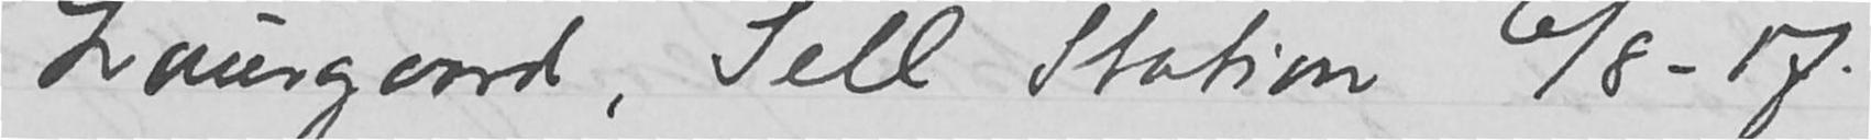Laurgaard, Sell Station 6/8 – 17.
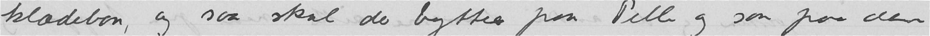klædebon, og saa skal der byttes paa Pelle og saa paa den
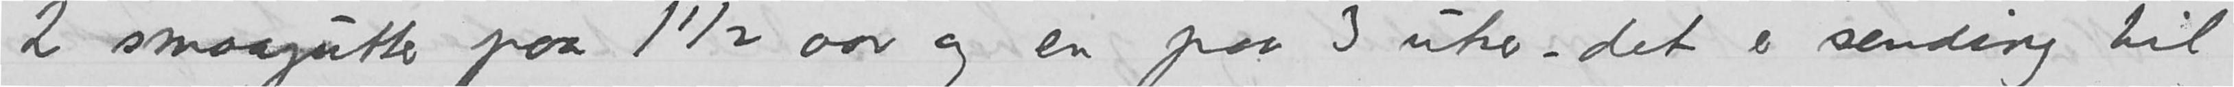2 smaagutter paa 1 ½ aar og en paa 3 uker – det er sending til
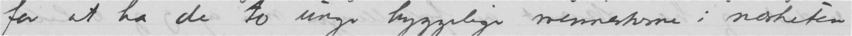for at ha de to unge hyggelige menneskene i nærheten
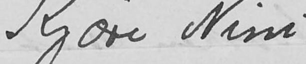Kjære Nini
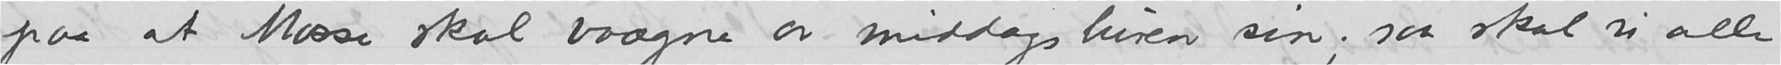paa at Mosse skal vaagne av middagsluren sin; saa skal vi alle
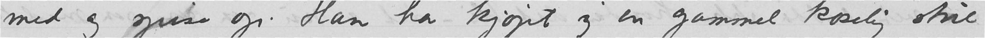med og spise op.  Han har kjøpt sig en gammel koselig stue
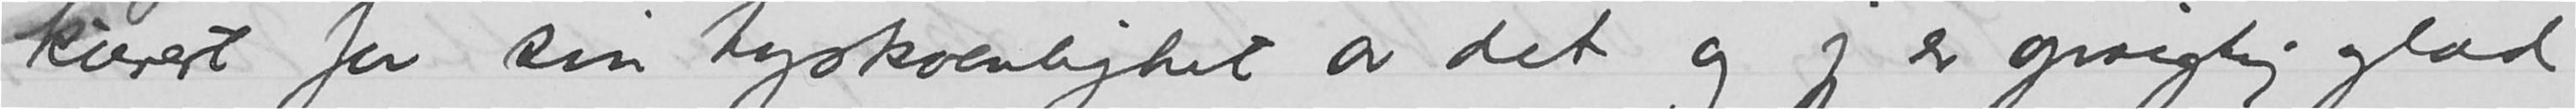kurert for sin tyskvenlighet av det og jeg er oprigtig glad
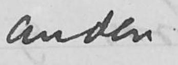anden.
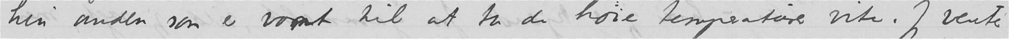hin anden som er vant til at ta de høie temperaturer vite. Jeg venter
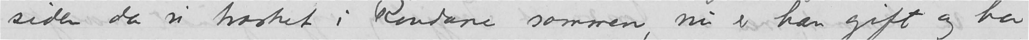siden da vi trasket i Rondane sammen, nu er han gift og har
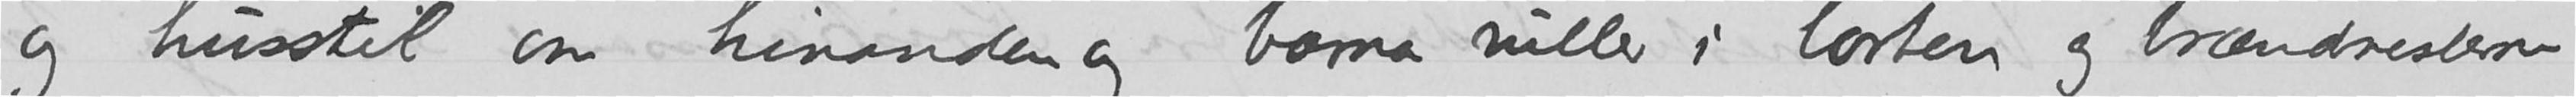og husstel om hinanden og barna ruller i lorten og brændneslerne
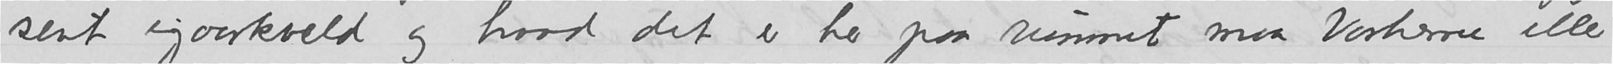sent igaarkveld og hvad det er her paa rummet maa Vorherre eller
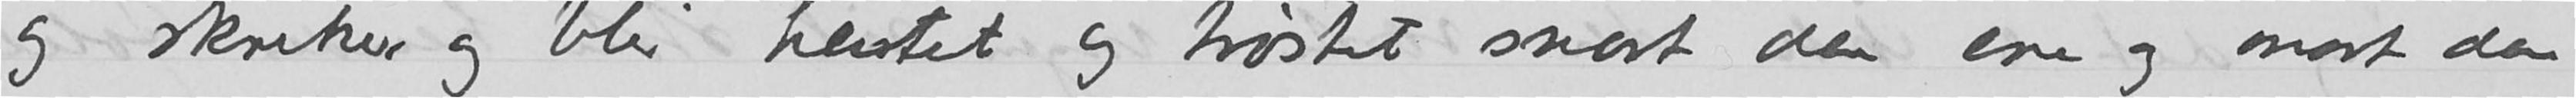og skriker og blir hentet og trøstet snart den ene og snart den
